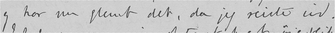og har nu glemt det, da jeg reiste ind.
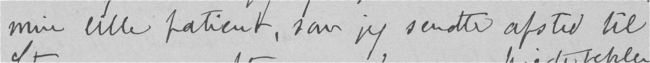min lille patient, som jeg sendte afsted til
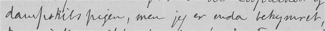dampskibspigen, men jeg er enda bekymret,
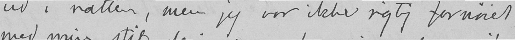ud i natten, men jeg var ikke rigtig fornøiet
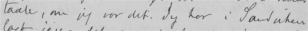taale, om jeg var det. Jeg har i Sandviken
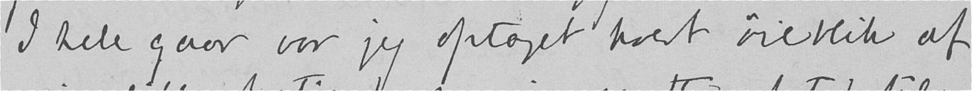I hele gaar var jeg optaget hvert øieblik af
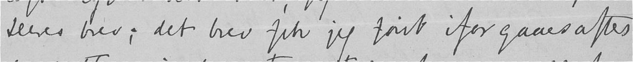Deres brev; det brev fik jeg først iforgaars aftes
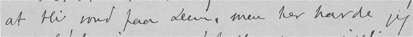at bli vond paa Dem, men her havde jeg
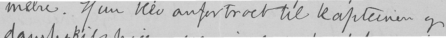melse. Hun blev anfortroet til kapteinen og
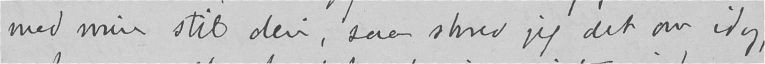med min stil deri, saa skrev jeg det om idag,
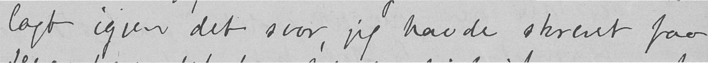lagt igjen det svar, jeg havde skrevet paa
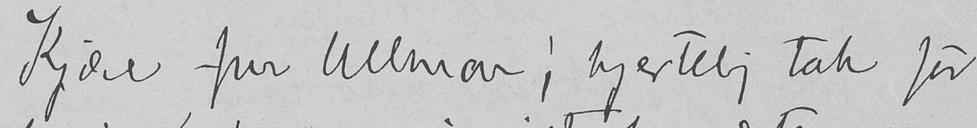Kjære fru Ullman; hjertelig tak for
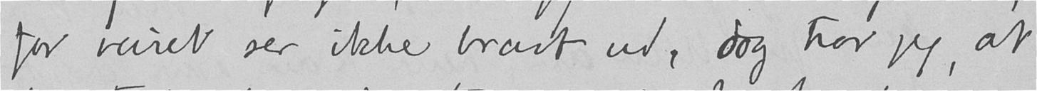for veiret ser ikke bravt ud, dog tror jeg, at
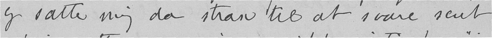og satte mig da strax til at svare sent
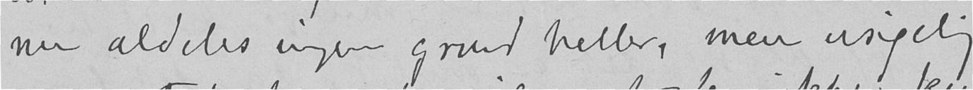nu aldeles ingen grund heller, men usigelig
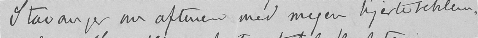Stavanger om aftenen med megen hjertebeklem-
